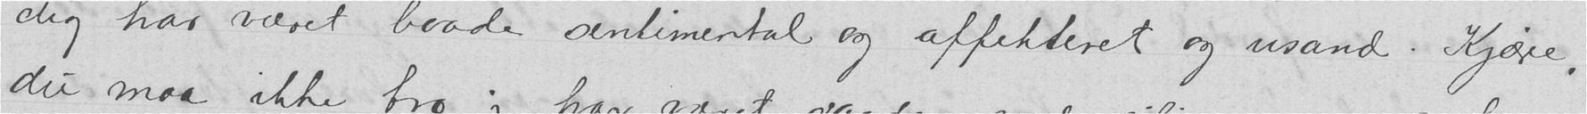dig har været baade sentimental og affekteret og usand. Kjære,
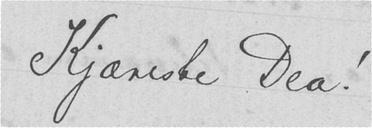Kjæreste Dea!
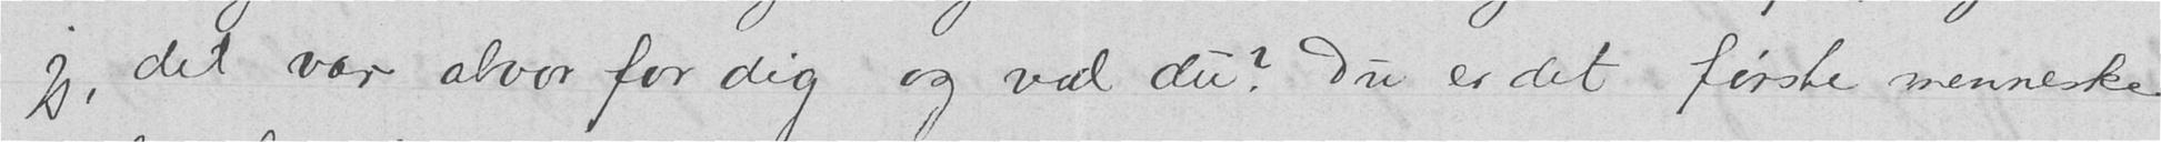jeg, det var alvor for dig og ved du? Du er det første menneske
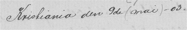Kristiania den 9de mai - 03.
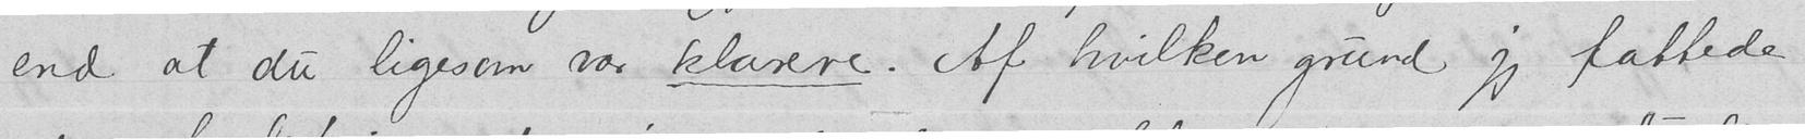end at du ligesom var klarere. Af hvilken grund jeg fattede
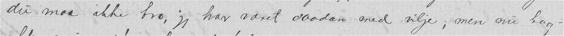du maa ikke tro, jeg har været saadan med vilje; men nu bag-
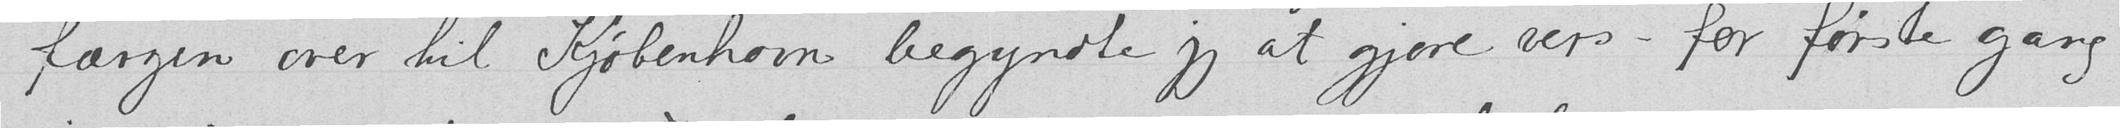færgen over til Kjøbenhavn begyndte jeg at gjøre vers - for første gang
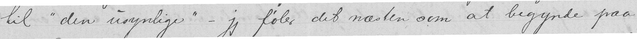til "den usynlige" - jeg føler det næsten, som at begynde paa
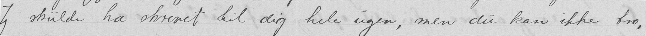Jeg skulde ha skrevet til dig hele ugen, men du kan ikke tro,
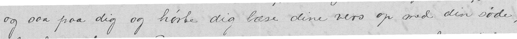og saa paa dig og hørte dig læse dine vers op med din søde,
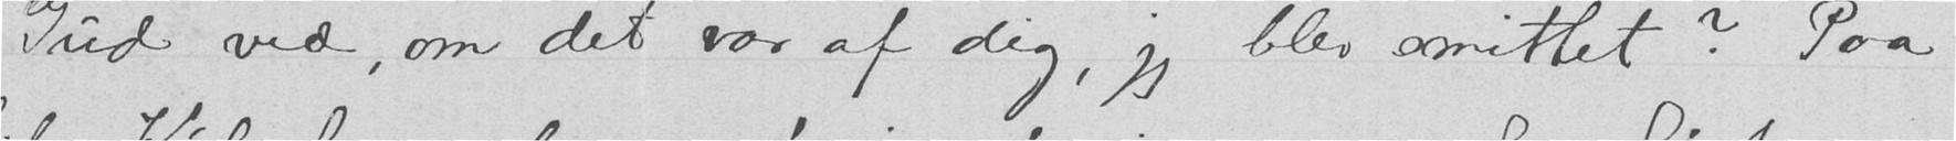Gud ved, om det var af dig, jeg blev smittet? Paa
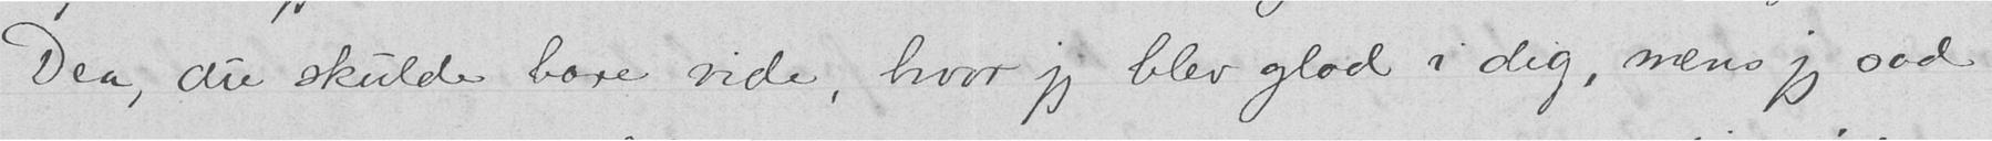Dea, du skulde bare vide, hvor jeg blev glad i dig, mens jeg sad
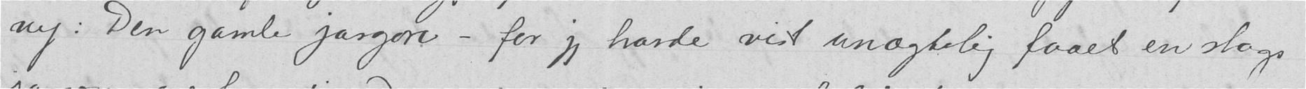ny. Den gamle jargon – for jeg havde vist unægtelig faaet en slags
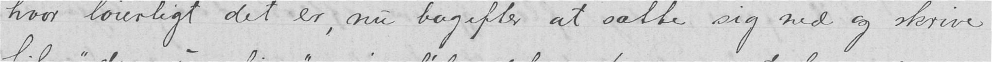hvor løierligt det er, nu bagefter at sætte sig ned og skrive
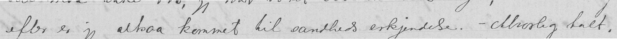efter er jeg altsaa kommet til sandheds erkjendelse. - Alvorlig talt,
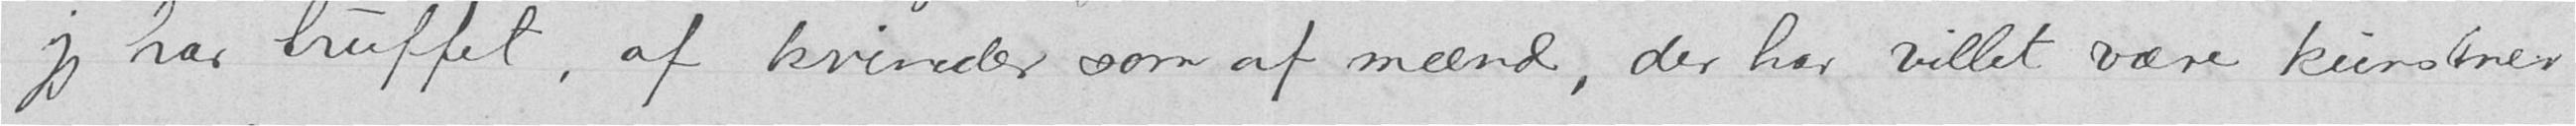jeg har truffet, af kvinder som af mend, der har villet være kunstner
